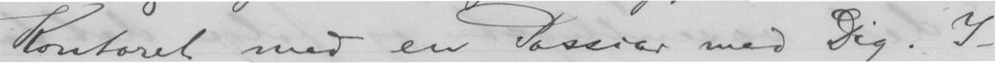Kontoret med en Passiar med Dig. I-
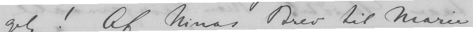get! Af Ninas Brev til Marie
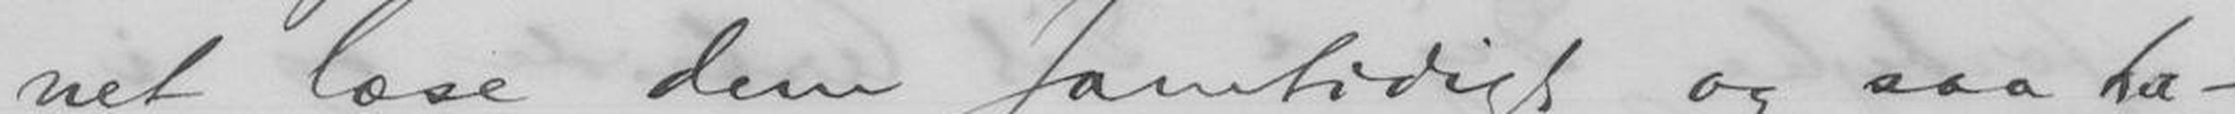net læse dem samtidigt og saa ha-
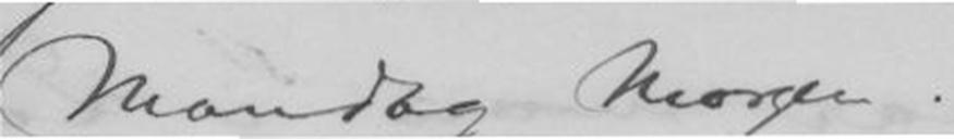Mandag Morgen.
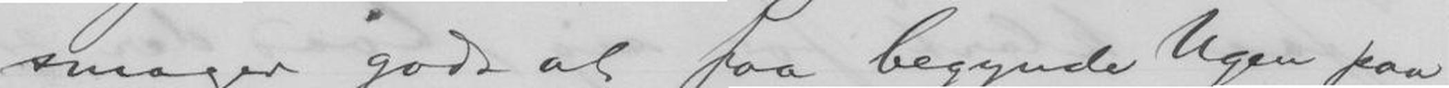smager godt at faa begynde Ugen paa
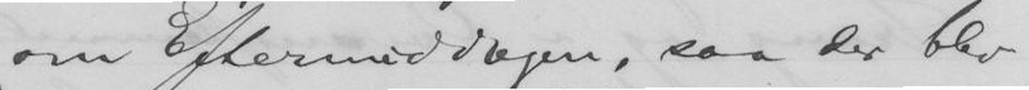om Eftermiddagen, saa der blev
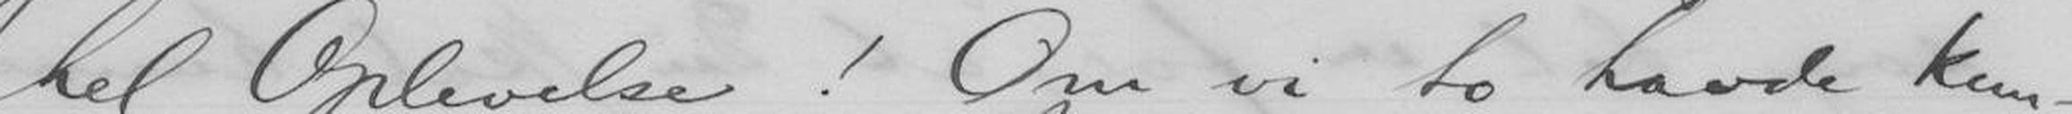hel Oplevelse! Om vi havde kun-
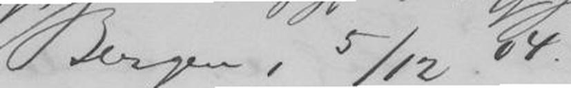Bergen, 15/12 04
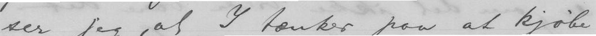ser jeg, at I tænker paa at kjøbe
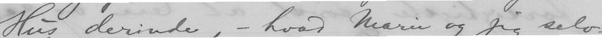Hus derinde, - hvad Marie og jeg selv-
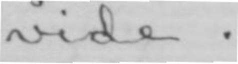vide.
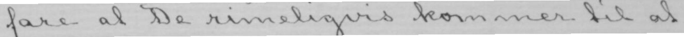fare at De rimeligvis kommer til at
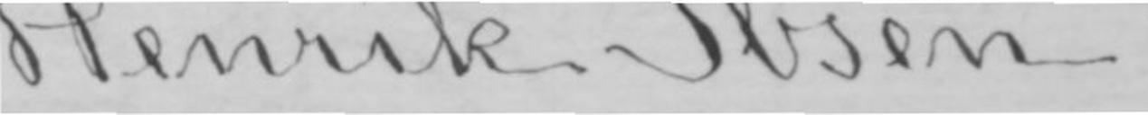Henrik Ibsen.
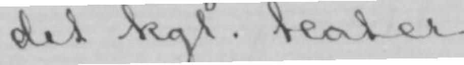det kgl. teater.
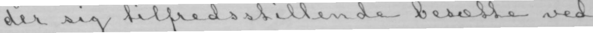der sig tilfredsstillende besætte ved
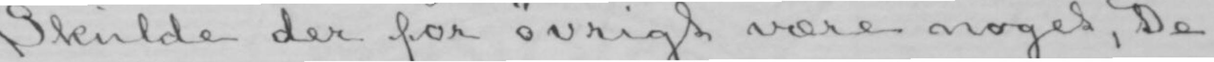Skulde der for øvrigt være noget, De
